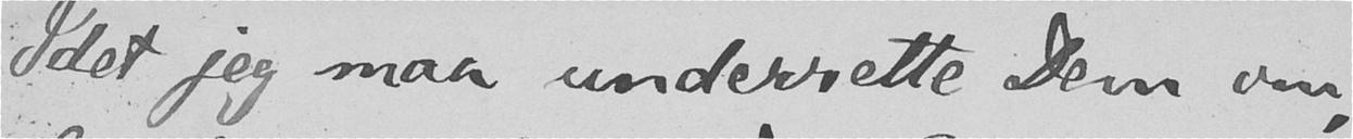Idet jeg maa underrette Dem om,
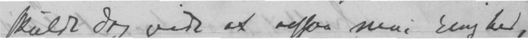skulde dog vide at ogsaa min Enighed,
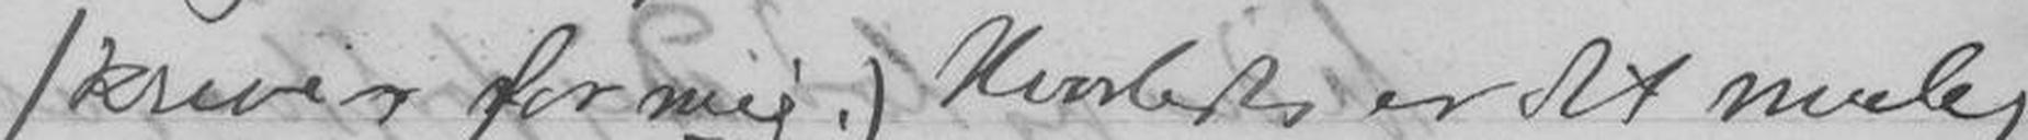skriverformig.) Hvorledes er det mulig
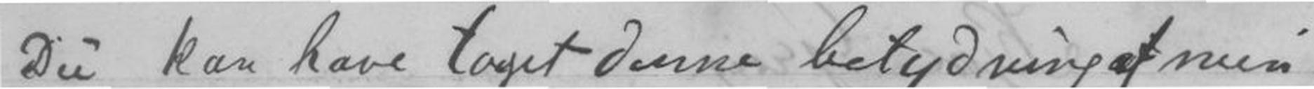Du kan have taget denne betydning af min
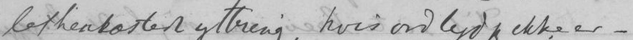lethenkastede yttring, hvis ordlyd jeg ikke er-
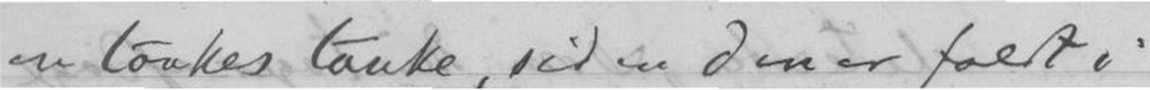en tankes tanke, siden den er faldt i
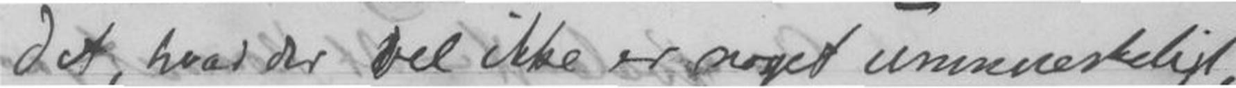det, hvad der vel ikke er noget umenneskeligt,
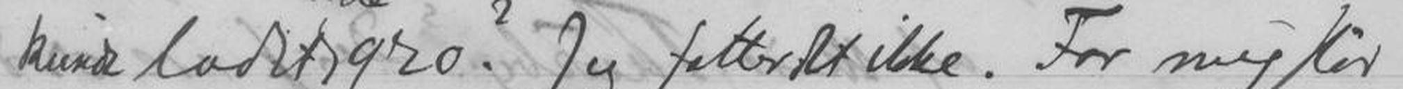kunde ladet gro? Jeg fatter det ikke. For mig stod
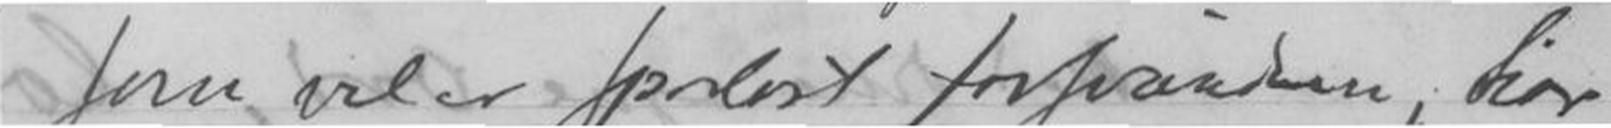som vel er sporløst forsvunden, har
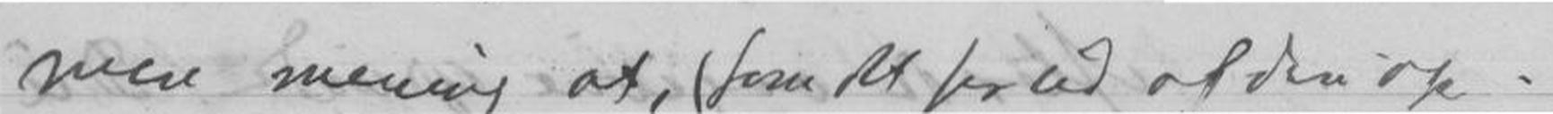min mening at, (som det ser ud af din op-
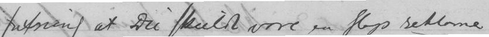fatning at Du skulde være en slags reklame-
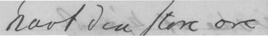havt den store ære
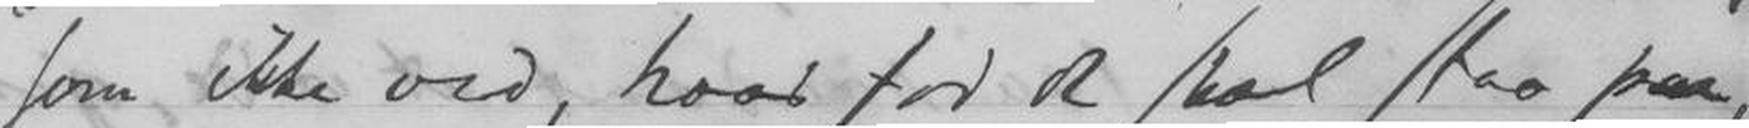som ikke ved, hvorfor de skal staa paa,
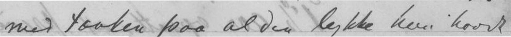med tanken paa al den lykke hun havde
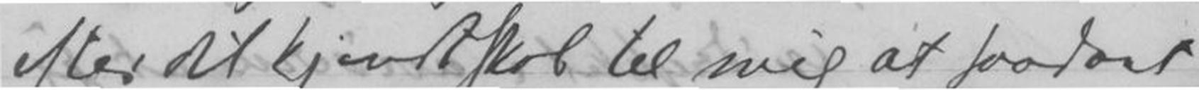efter det kjendtskab til mig at saadant
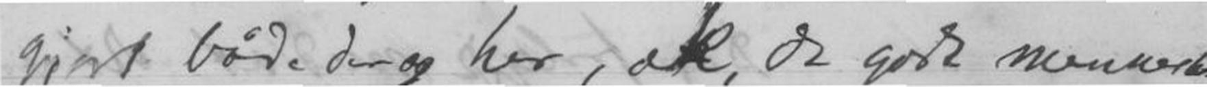gjort både der og her, ak, de gode menneske
